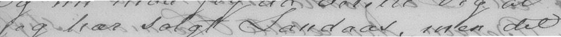jeg har solgt Landaas, men det
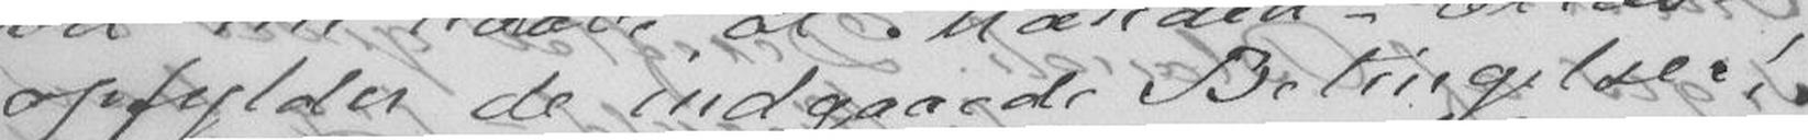opfylder de indgaaede Betingelse!
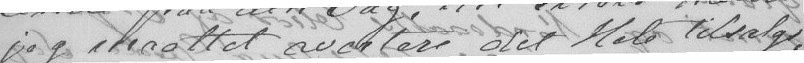jeg maattet avertere det Hele tilsalgs,
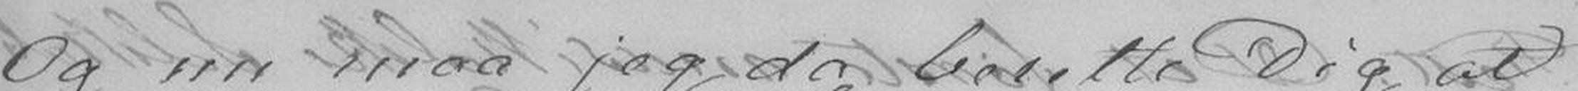Og nu maa jeg da berette Dig at
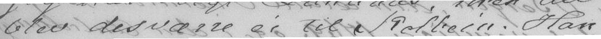blev desværre ei til Kolbein. Han
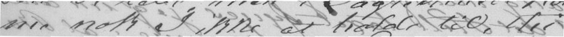me nok I ikke at holde til, thi
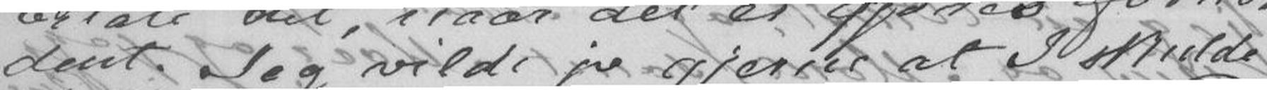dent. Jeg vilde jo gjerne at I skulde
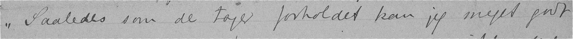"Saaledes som de tager forholdet kan jeg meget godt
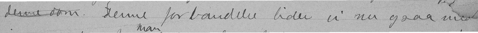denne dom. Denne forbandelse lider vi nu ogsaa med
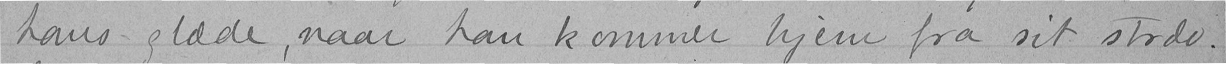hans glæde, naar han kommer hjem fra sit stræv.
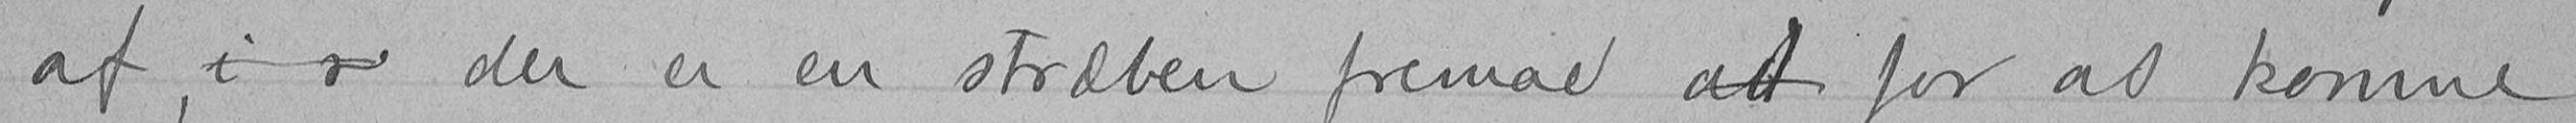af, i re der er en stræben fremad at for at komme
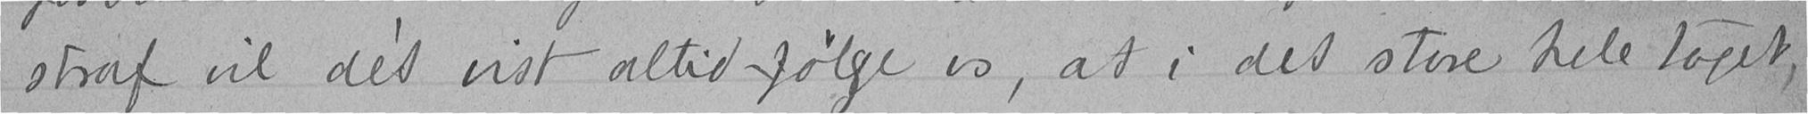straf vil det vist altid følge os, at i det store hele taget,
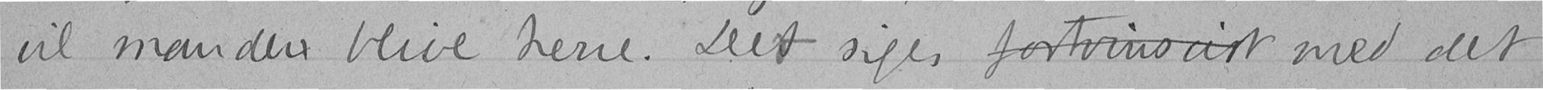vil manden blive herre. Det siges fortrinsvist med det
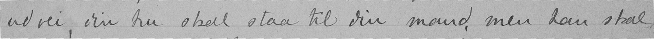udvei, din hu skal staa til din mand, men han skal
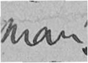man
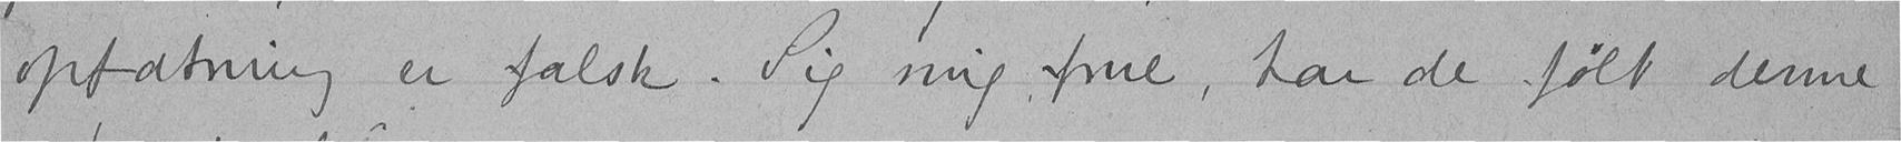opfatning er falsk. Sig mig frue, har de følt denne
